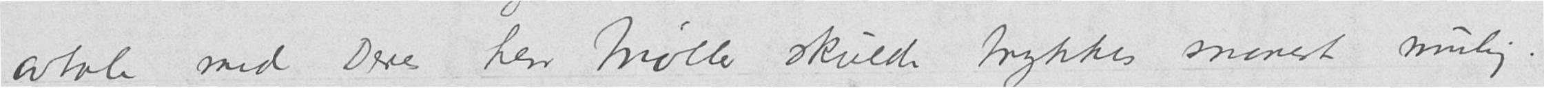avtale med Deres herr Møller skulde trykkes snarest mulig.
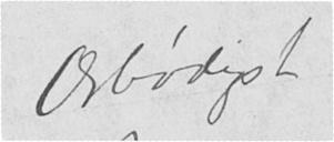Ærbødigst
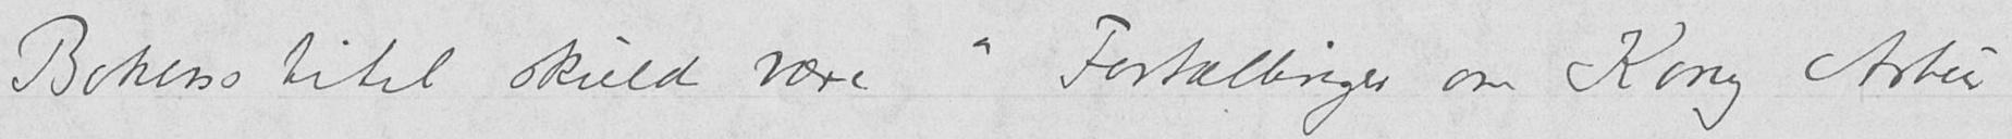Bokens titel skulde være "Fortællinger om Kong Artur
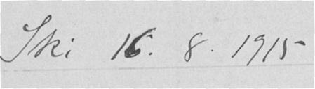Ski 16.8.1915
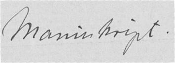Manuskript.
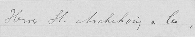Herrer H. Aschehoug & Co,
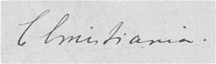Christiania.
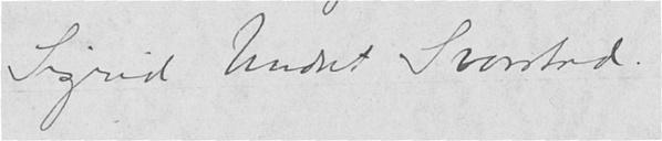Sigrid Undset Svarstad.
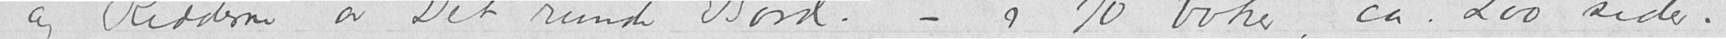og Ridderne av Det runde Bord." - i to bøker, ca. 200 sider.
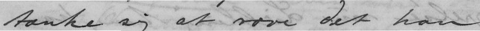tænke sig at vove det han
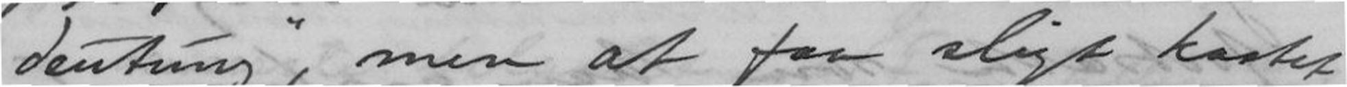deutung", men at faa sligt kastet
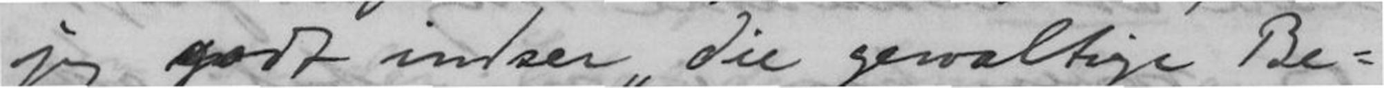jeg godt indser "die gewaltige Be-
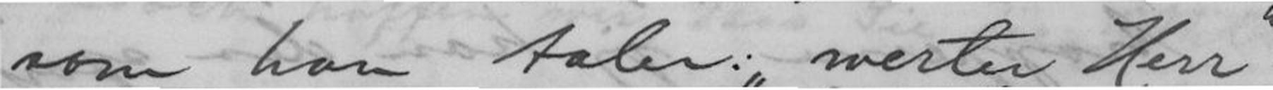som han taler:"werter Herr" -
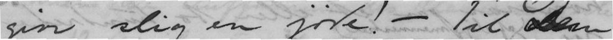give slig en jöde! - Til Dem
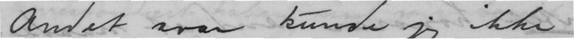Andet svar kunde jeg ikke
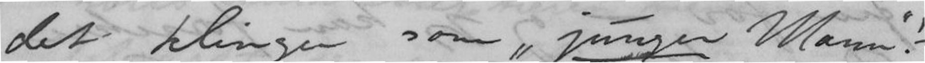det klinger som "jungen Mann"!-
